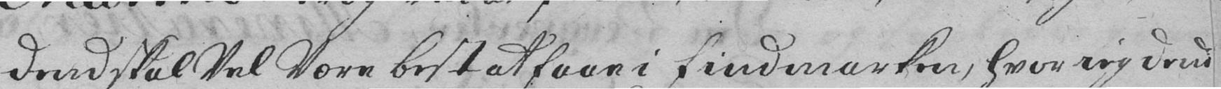dend skal Vel Være best at faae i Findmarken, hvor ieg dend
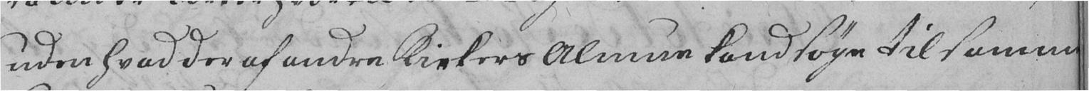uden hvad der af andre Kirkers Almue kand søge til samme
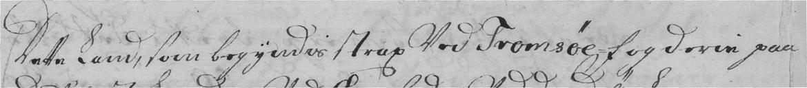Dette Land, som begyndis strax Ved Tromsøe-Fogderie paa
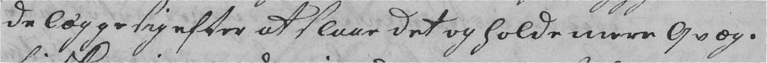de lægge sig efter at slaae det og holde mere Qvæg.
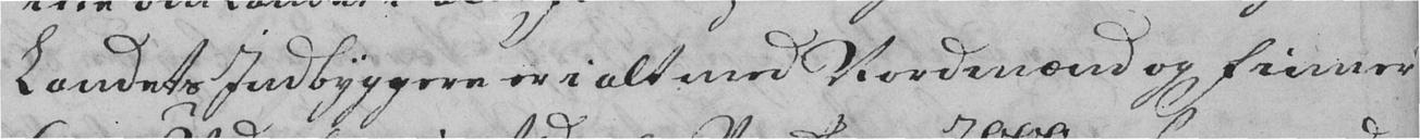Landets Indbyggere er i alt med Nordmænd og Finner
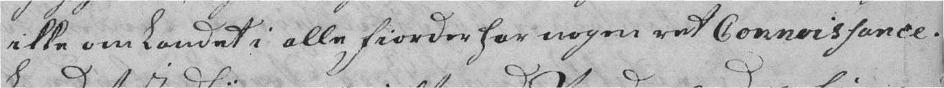ikke om Landet i alle Fiorder har nogen ret Connoissance.
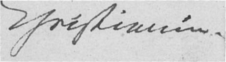Christiania.
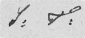S: T:
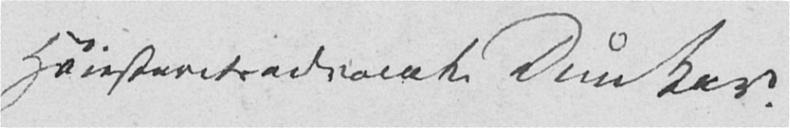Høiesteretsadvocat Dunker.
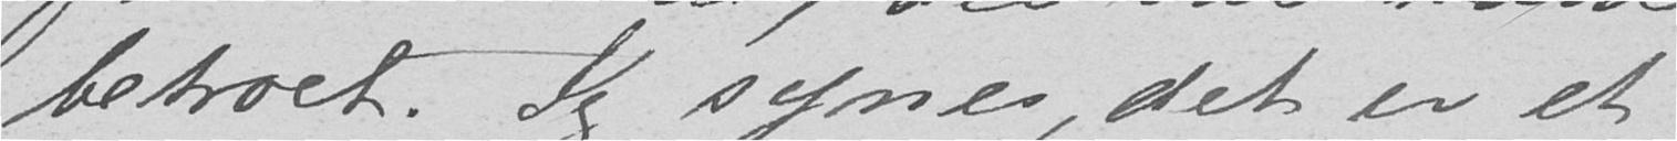betroet. Jeg synes det er et
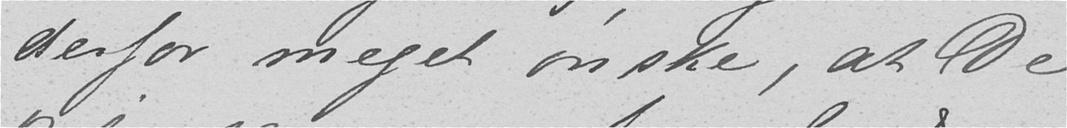derfor meget ønske, at De
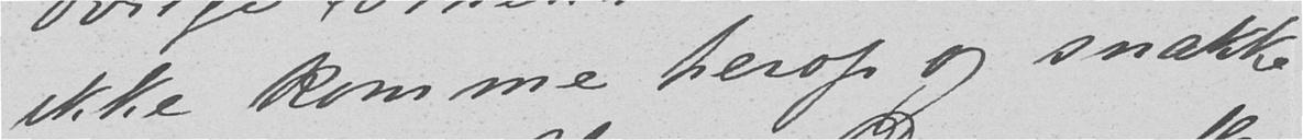ikke komme herop og snakke
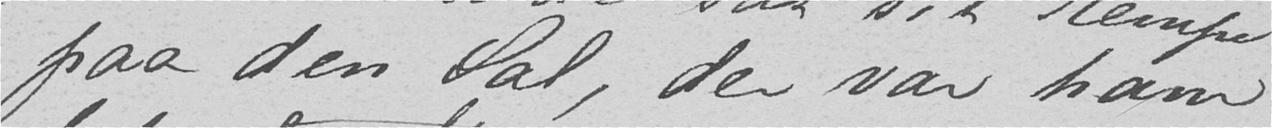paa den Sal, der var ham
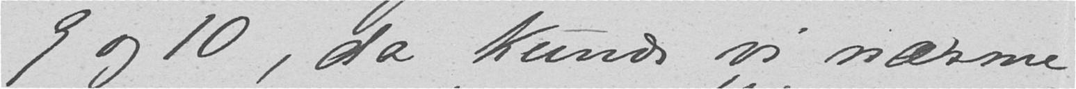9 og 10, da kunde vi nærme
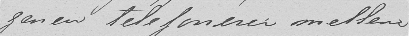genen Telefonerer mellem
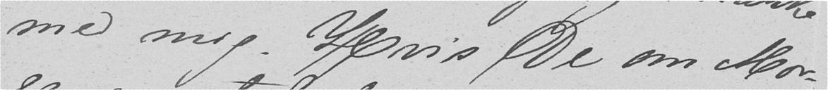med mig. Hvis De om Mor-
